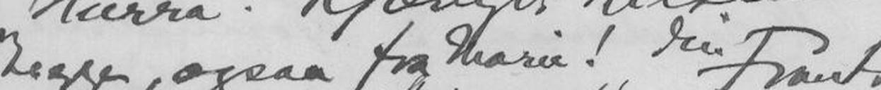Begge, ogsaa fra Marie! Din Frants.
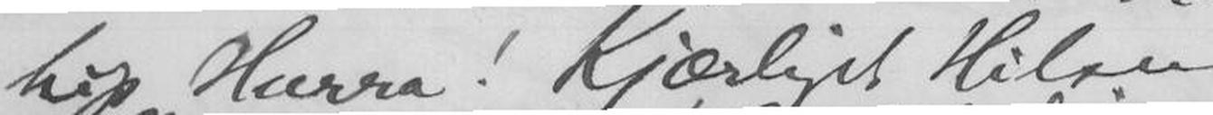hip Hurra! Kjærligst Hilsen
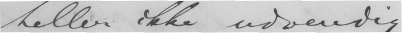heller ikke udvendig
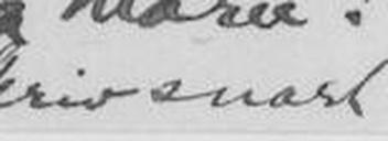Skriv snart!
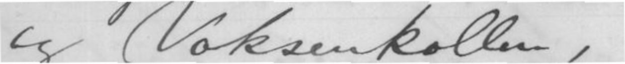og Voksenkollen, og
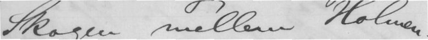Skogen mellem Holmen-
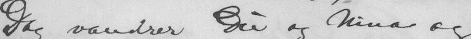Dag vandrer Du og Nina og
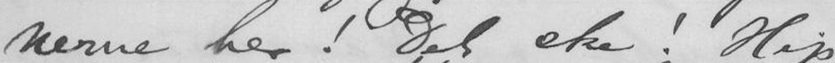nerne her! Det ske! Hip
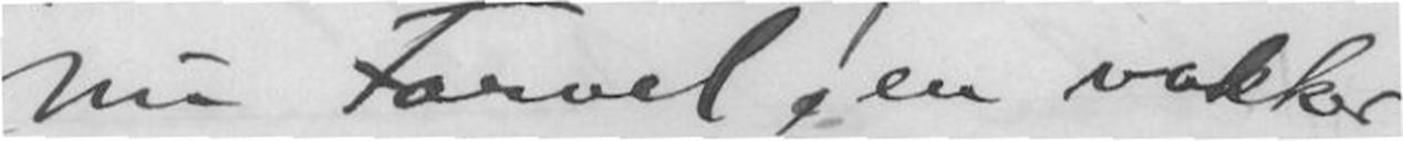Nu Farvel! en vakker
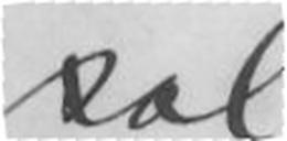sal!
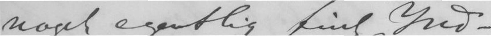noget egentlig fint Ind-
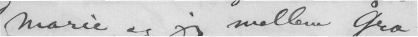Marie og jeg mellem Gra-
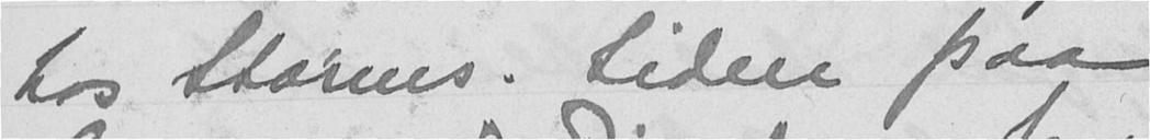hos Storms. Siden paa
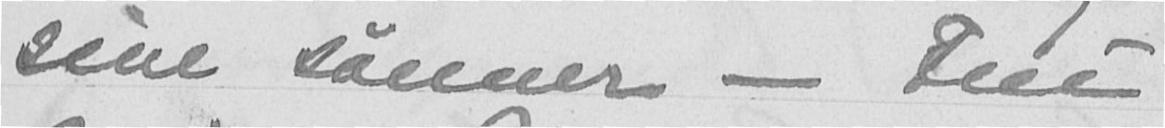sine sønner - Fru
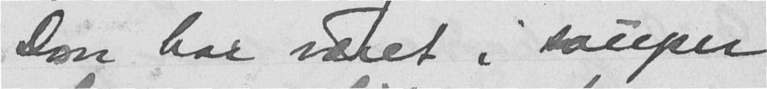Don har været i souper
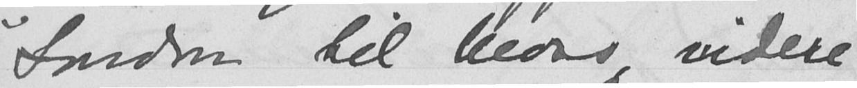London til Moss, videre
